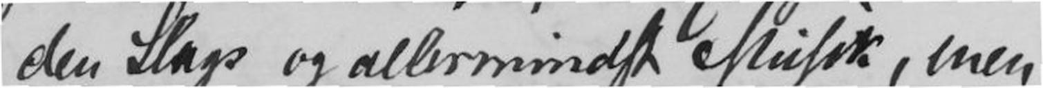den Slags og allermindst Musik, men
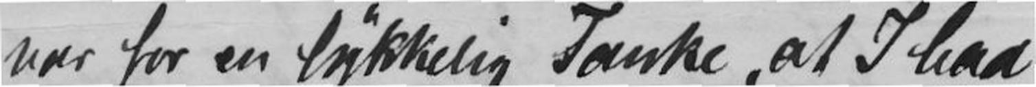var for en lykkelig Tanke, at I bad
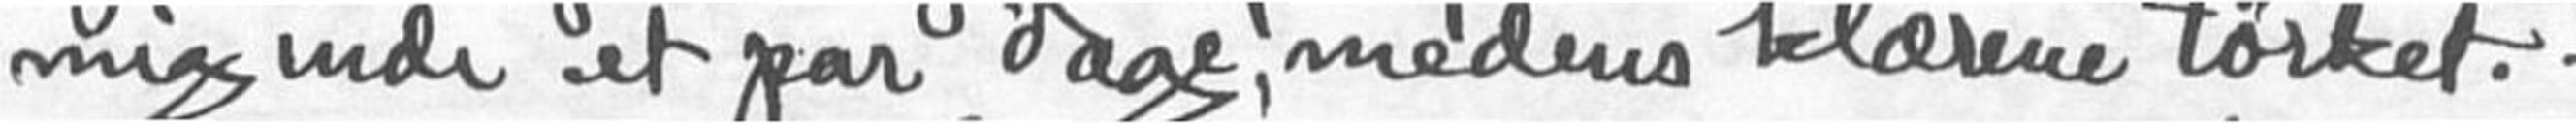mig inde et par dage, medens klærne tørket. -
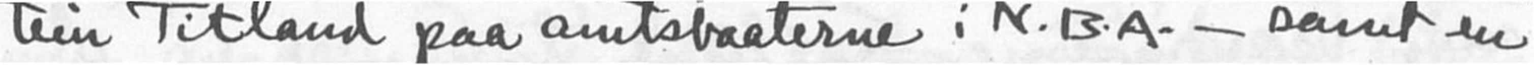tein Titland paa amtsbaaterne i N.B.A. - samt en
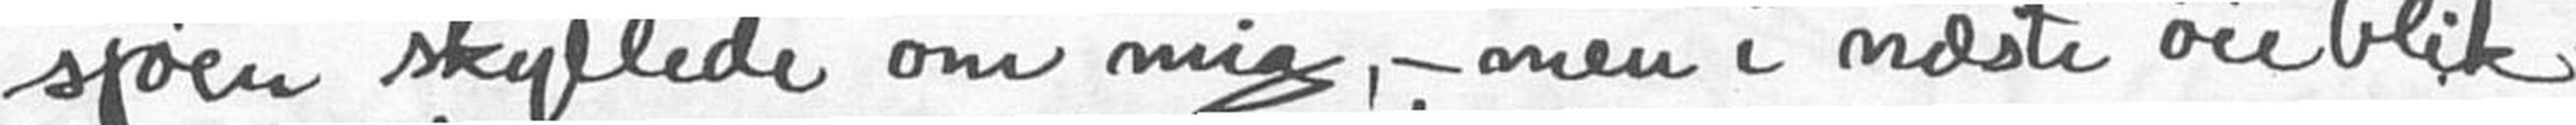sjøen skyllede om mig, - men i næste øieblik
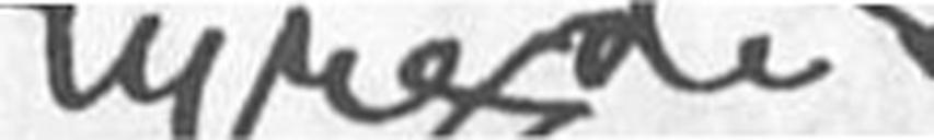tyngde
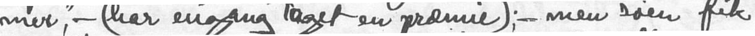mer" - (har engang taget en præmie): - men søen fik
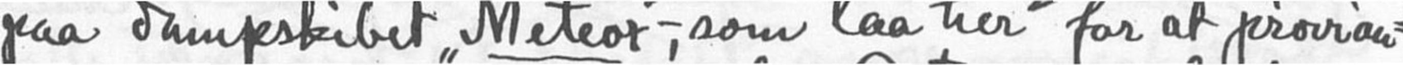paa dampskibet "Meteor" -, som laa her for at provian-
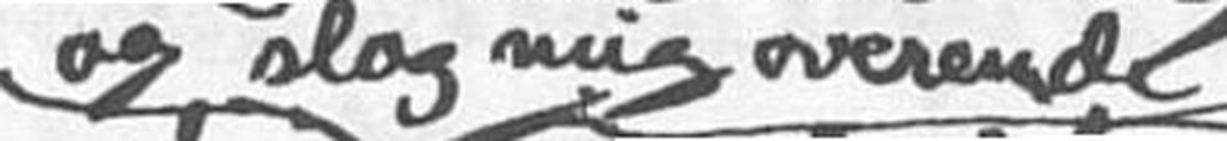og slog mig overende
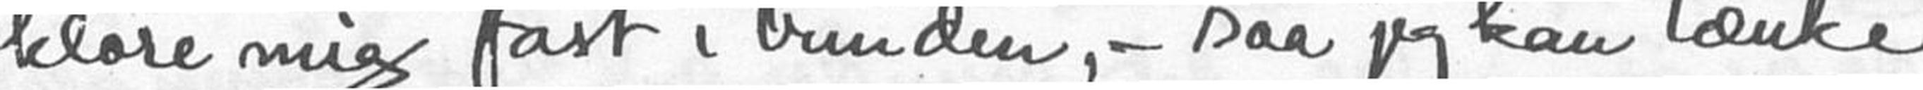klore mig fast i bunden, - saa jeg kan tænke
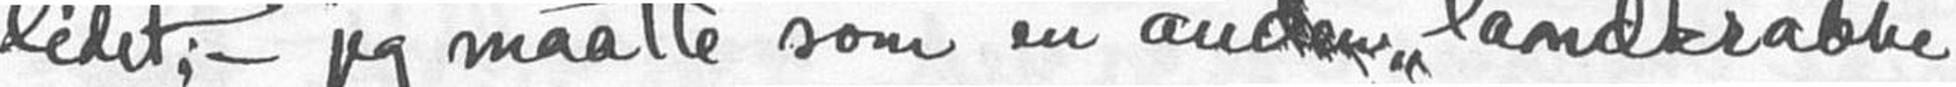lidet; - jeg maate som en anden "landkrabbe"
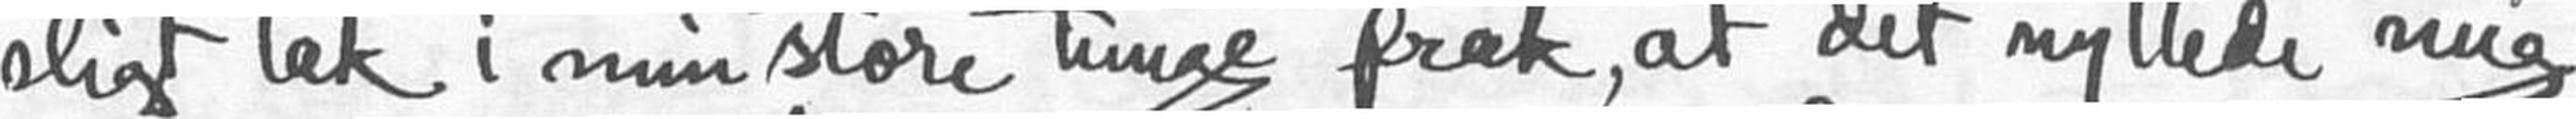sligt tak i min store tunge frak, at det nyttede mig
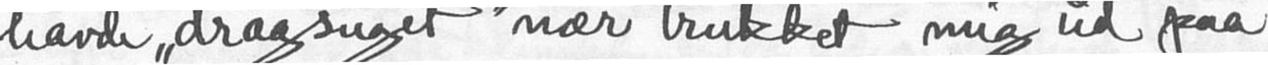havde "dragsuget" nær trukket mig ud paa
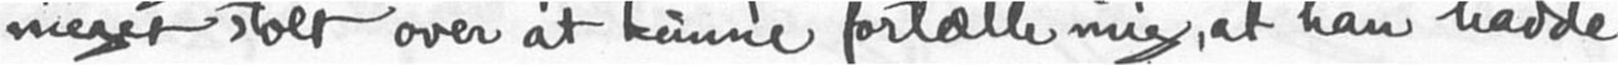meget stolt over at kunne fortælle mig, at han hadde
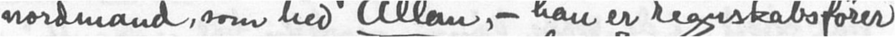nordmand, som hed Allan, - han er regnskabsfører
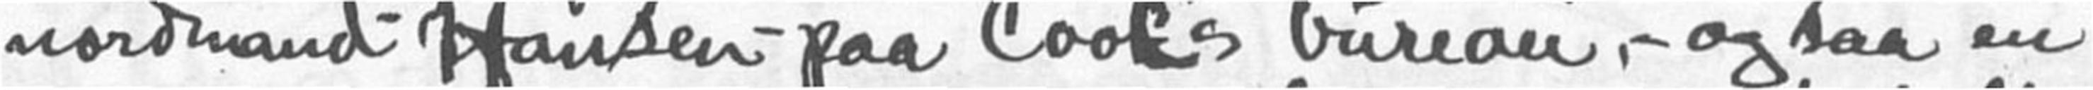nordmand - Hansen - paa Cook's bureau, - og saa en
